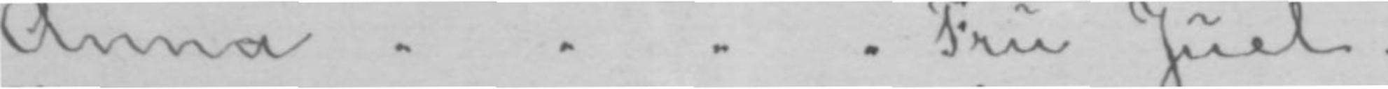Anna " " " " Fru Juel.
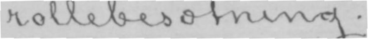rollebesætning.
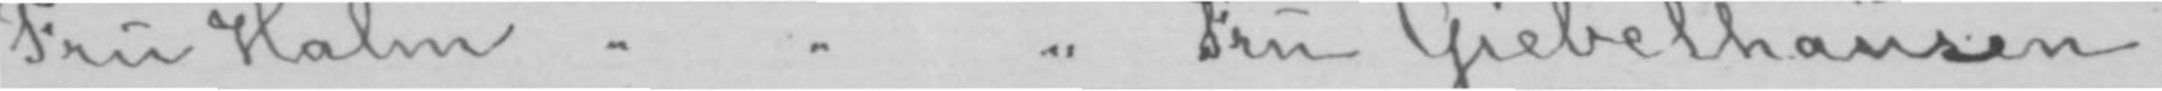Fru Halm " " " Fru Giebelhausen.
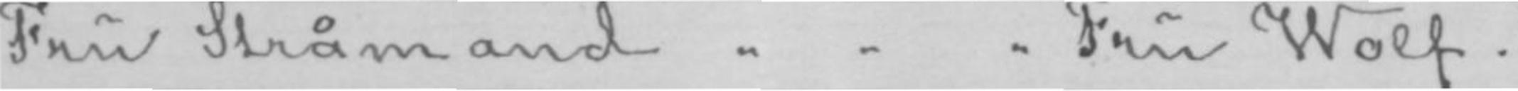Fru Stråmand " " " Fru Wolf.
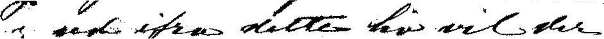og ud ifra dette Liv vil der
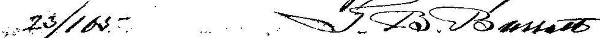23/1 05T. B. Barratt
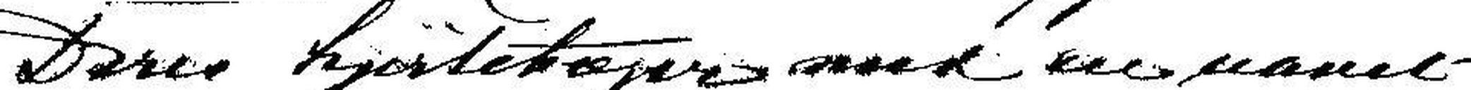Deres hjertetæger med en uanet
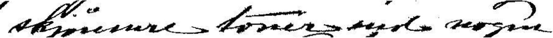skjønnere toner end nogen-
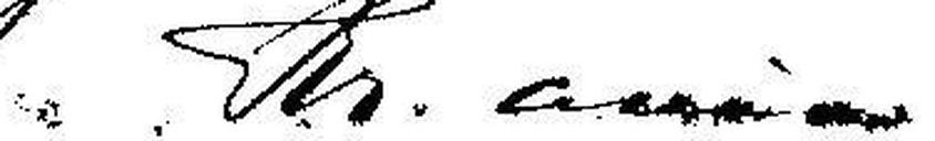Kr.ania
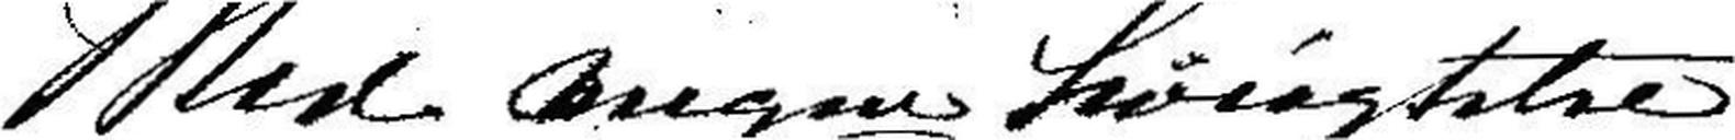Med megen høiagtelse
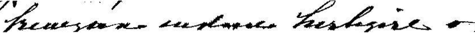fremgaa endnu herligere og
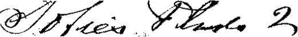Sofies Plads 2
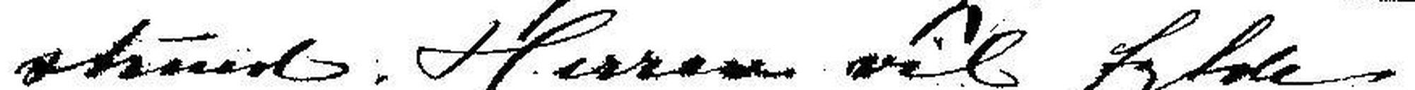stund. Herren vil fylde
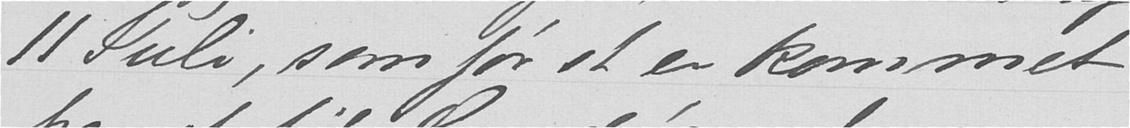11 Juli, som først er kommet
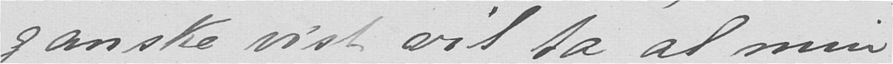ganske vist vil ta al min
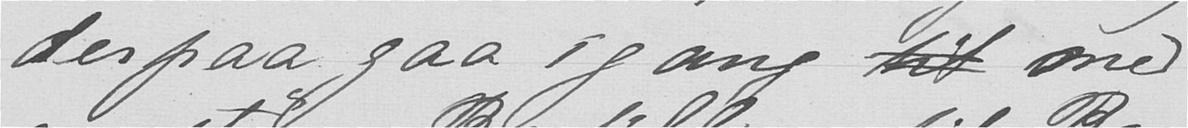derpaa gaa igang til med
